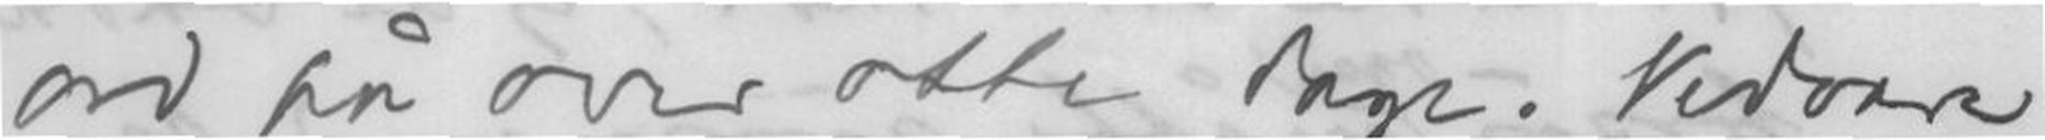ord på over otte dage. Vedvare
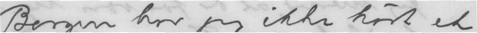Bergen har jeg ikke hørt et
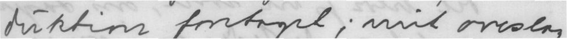duktion fortaget; mit overslag
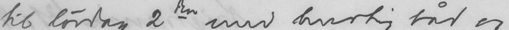til lørdag 2den med hurtig båd og
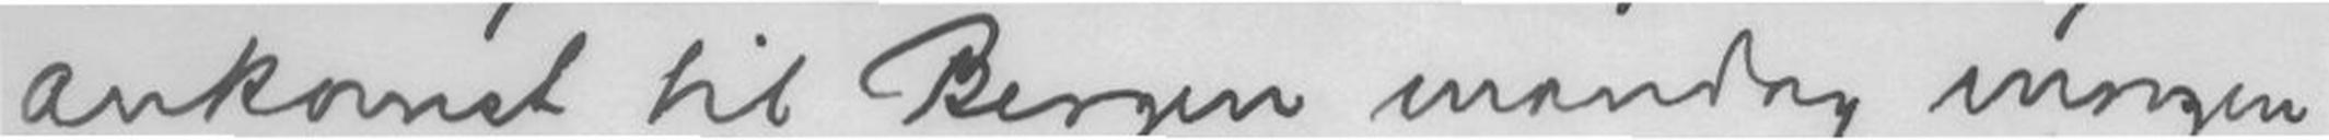ankomet til Bergen mandag morgen
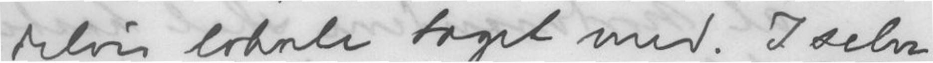delvis lokale taget med. I selve
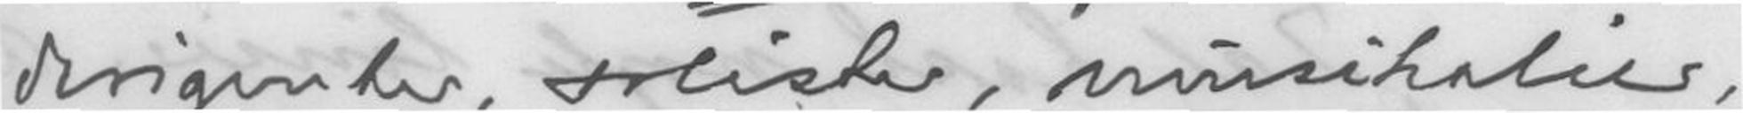dirigenter, solister, musikalier,
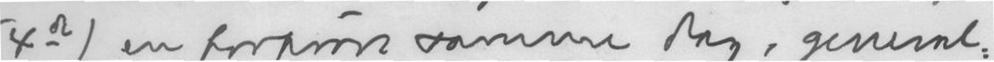(4de) en forprøve samme dag, general-
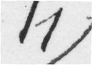11)
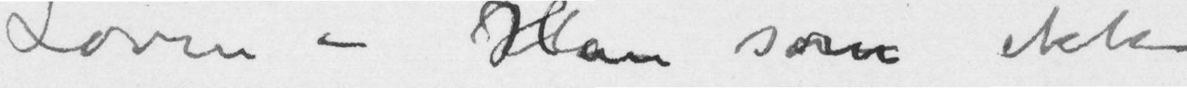Loven – Han som ikke
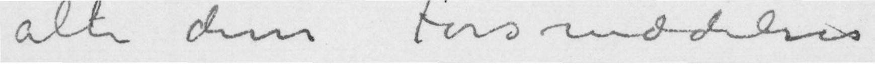alle disse Forsmædelser
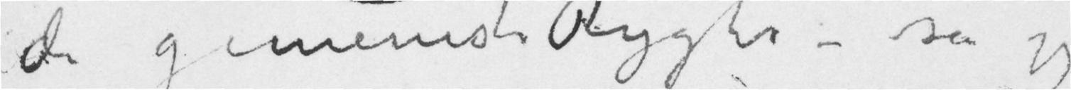de gemeneste Rygter – så jeg
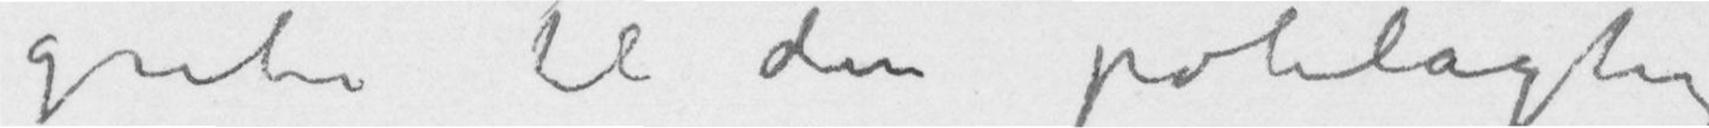gribe til den pøbelagtige
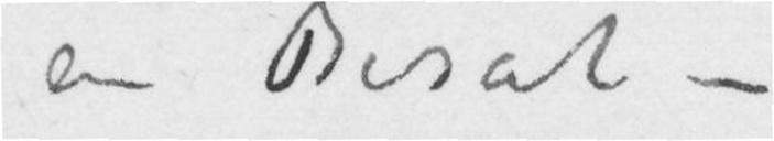en Besat –
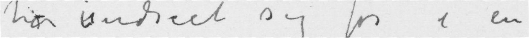har undseet sig for i en
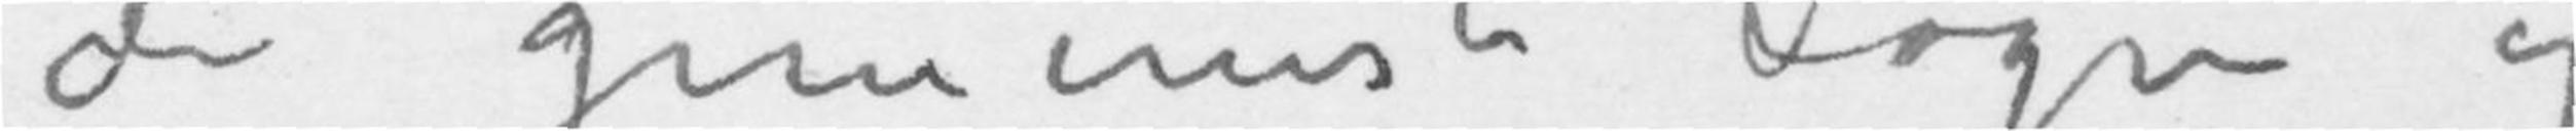de gemeneste Løgne og
